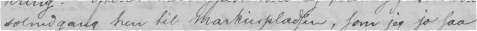solnedgang hen til Markuspladsen, som jeg jo saa
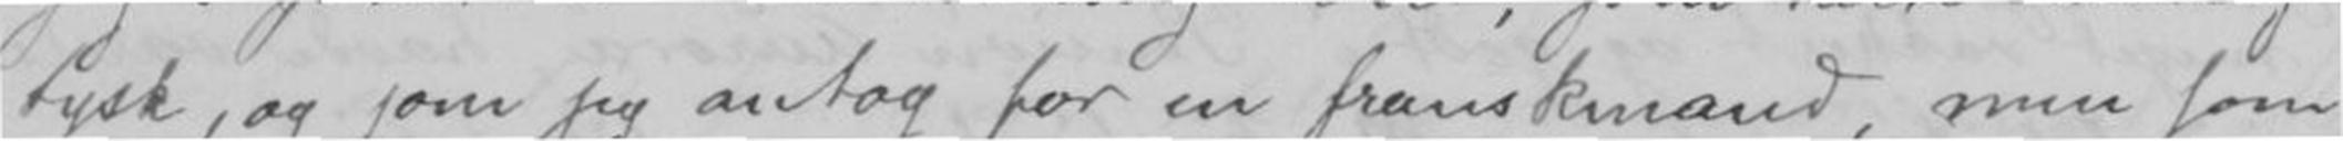tysk, og som jeg antog for en franskmand, men som
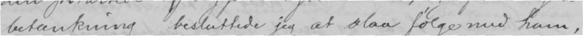betænkning besluttede jeg at slaa følge med ham,
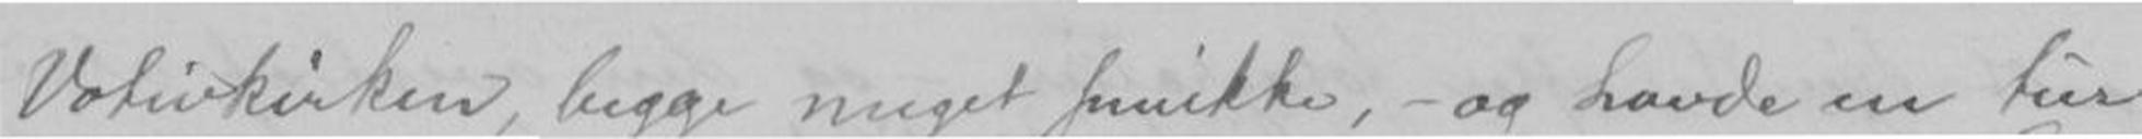Votivkirken, begge meget smukke,- og havde en tur
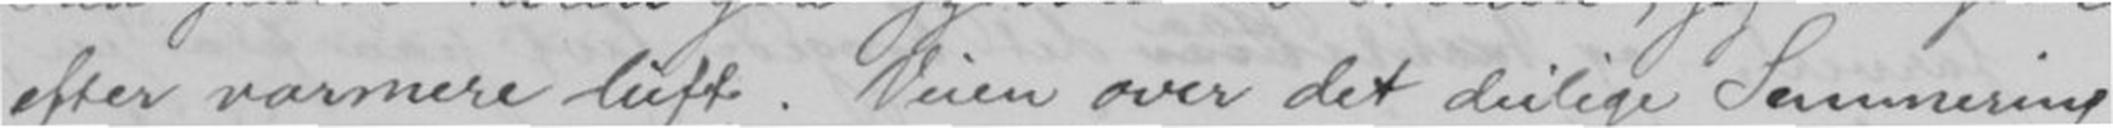after varmere luft. Veien over det deilige Sammering
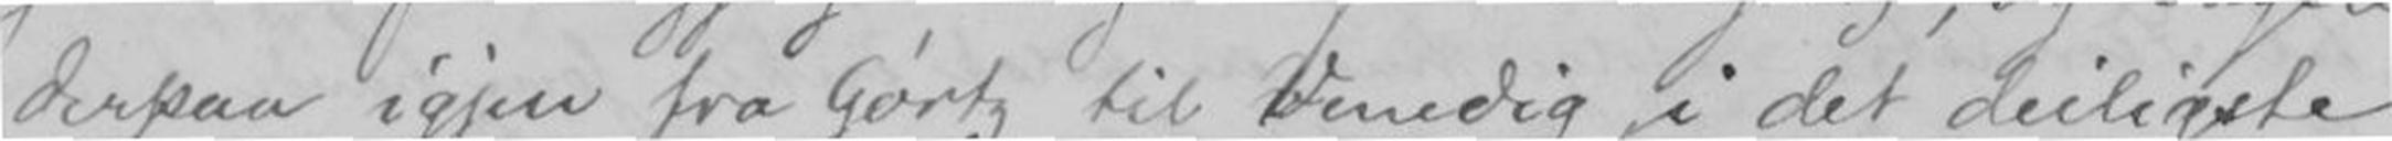derefter igjen fra Gortz til Venedig i det deiligste
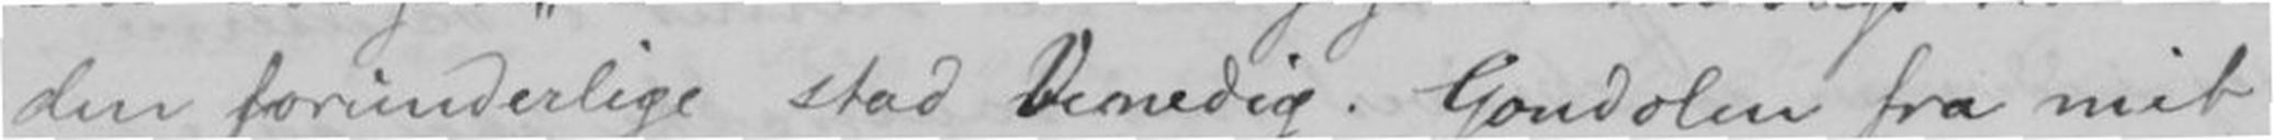den forunderlige stad Venedig. Gondolen fra mit
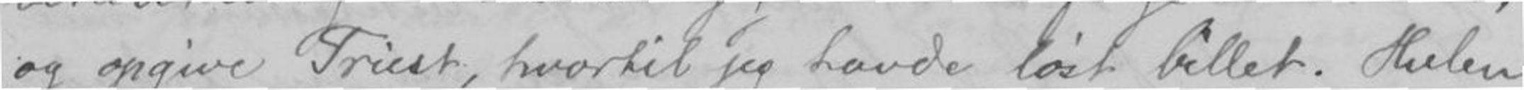og opgive Triest, hvortil jeg havde løst billet. Hulen
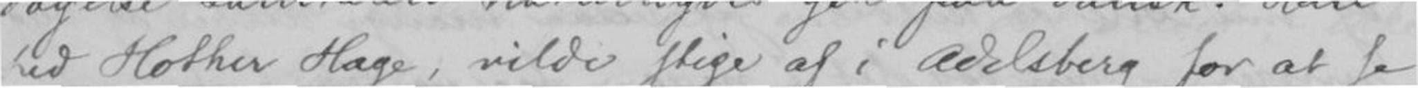hed Hother Hage, vilde stige af i Adelsberg for at se
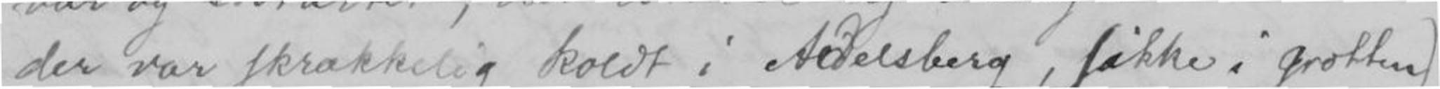der var skrækkelig koldt i Adelsberg, (ikke i grotten)
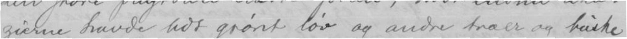zierne havde lidt grønt løv og andre træer og buske
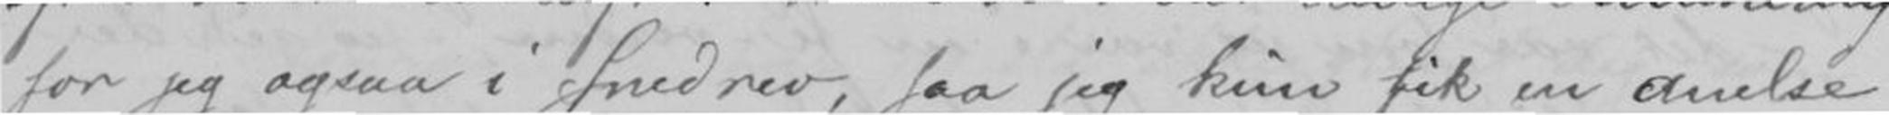for jeg ogsaa i Snedrev, saa jeg kun fik en anelse
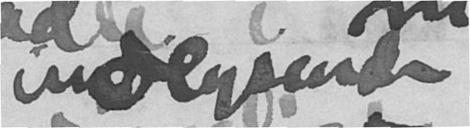indlysende
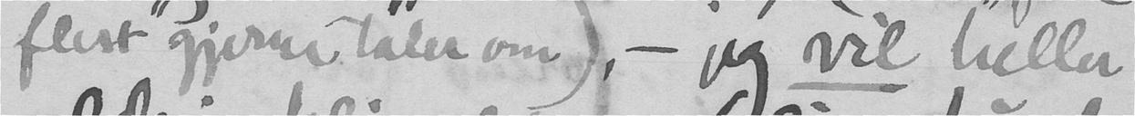flest gjerne taler om), - jeg vil heller
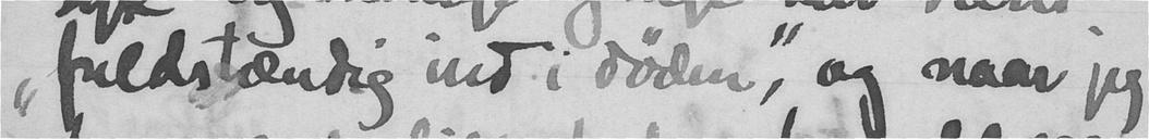"fuldstændig ind i døden", og naar jeg
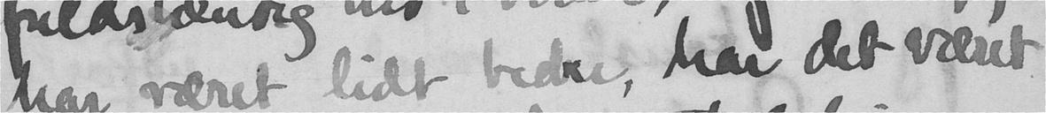har været lidt bedre, har det været
